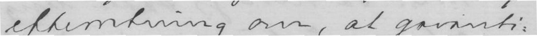efterretning om, at garanti-
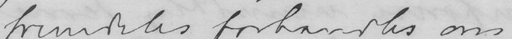fremdeles forhandles om
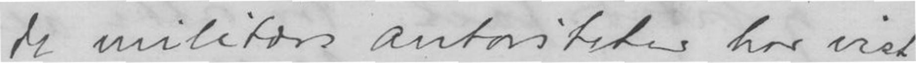de militære autoriteter har vist
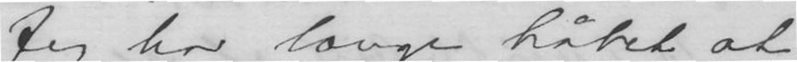Jeg har længe håbet at
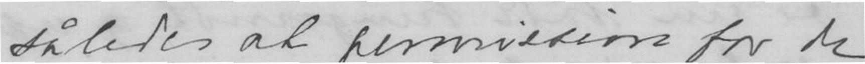således at permission for de
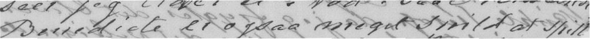Benedicte er ogsaa meget snild at spill
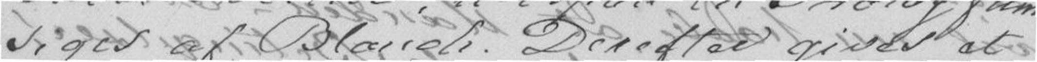siges af Blanch. derefter gives et
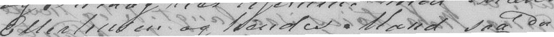Ellerhusen og hendes Mand, saa Du
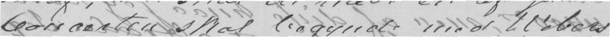Concerten skal begynde med Webers
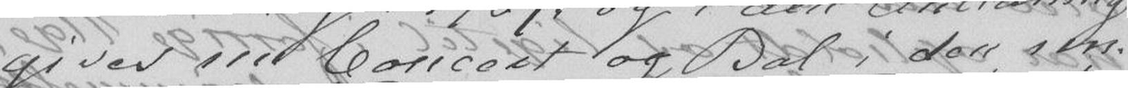gives nu Concert og Bal i den nu-
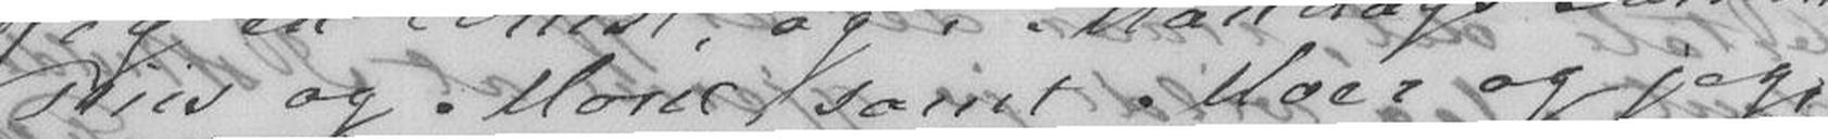Riis og Mone, samt Moer og jeg,
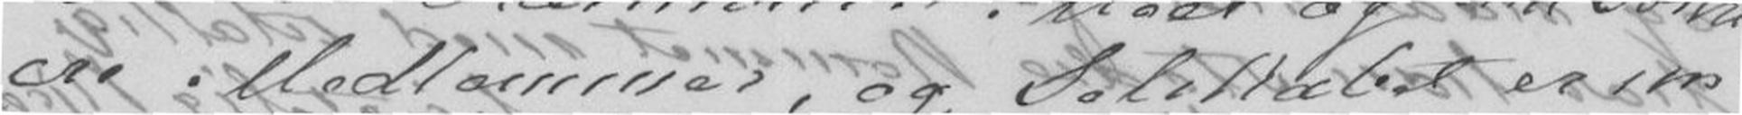ere Medlemmer, og Selskabet er nu
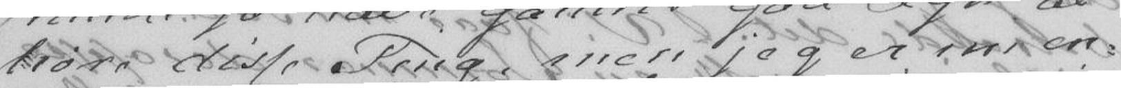høre disse Ting, men jeg er nu en-
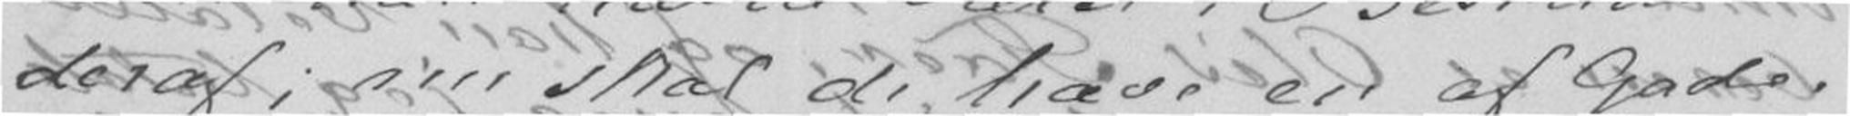deraf; nu skal de have en af Gade.
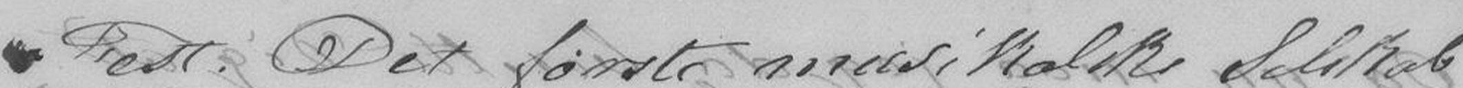Fest. Det første musikalske Selskab
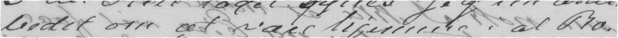bedst om at være hjemme i al Ro.
